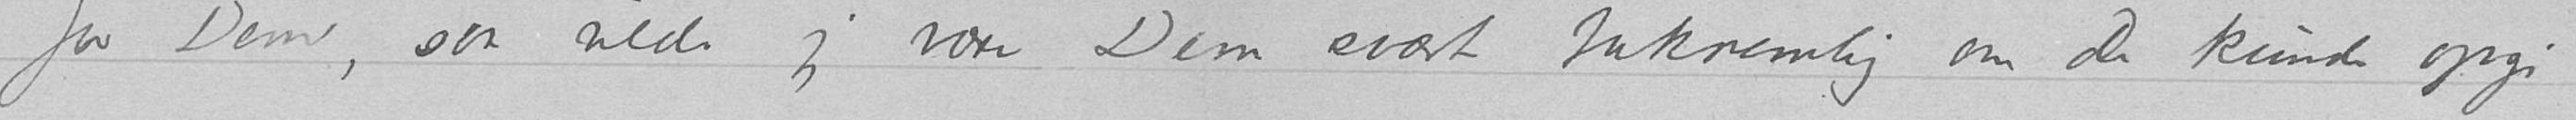for Dem, saa vilde jeg være Dem svært taknemlig om de kunde opgi
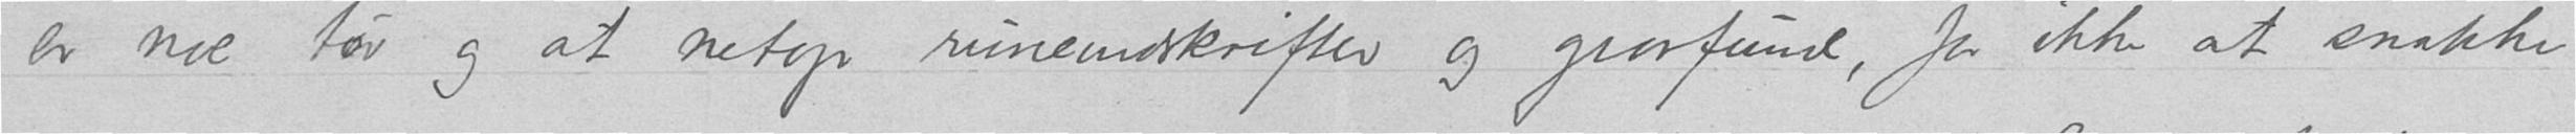er noe tøv og at netop runeindskrifter og gravfund, for ikke at snakke
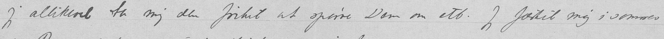jeg allikevel ta mig den frihet at spørre Dem om ett. Jeg fæstet mig i sommer
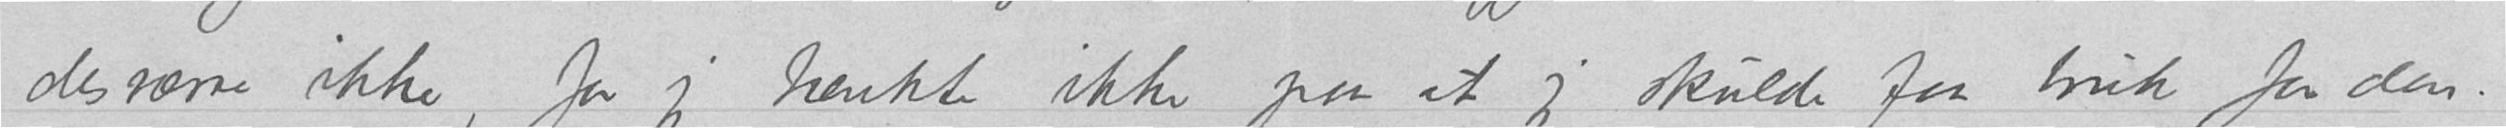desværre ikke, for jeg tænkte ikke paa at jeg skulde faa bruk for den.
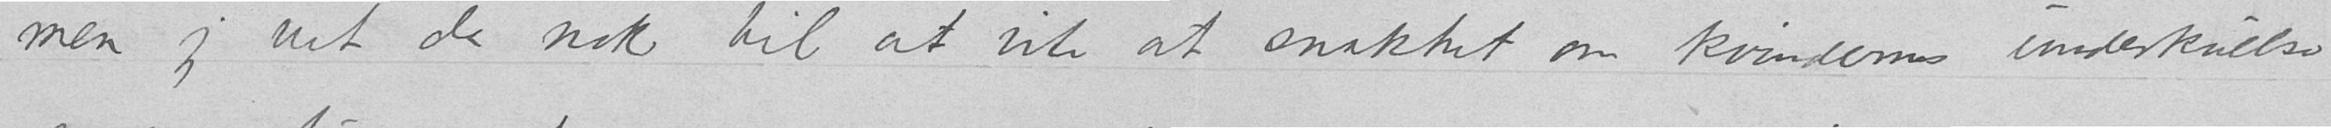men jeg vet da nok til at vite at snakket om kvindernes underskuelse
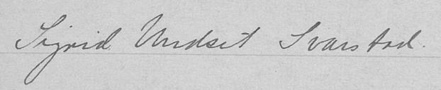Sigrid Undset Svarstad.
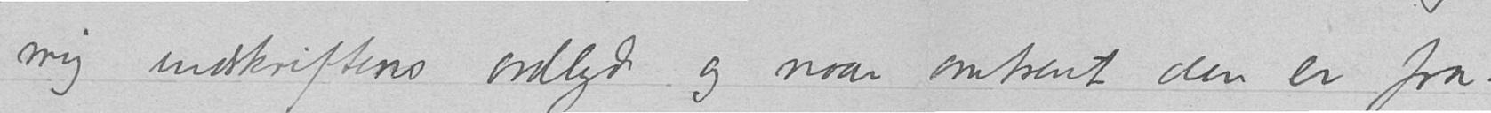mig indskriftens ordlyd og naar omtrent den er fra.
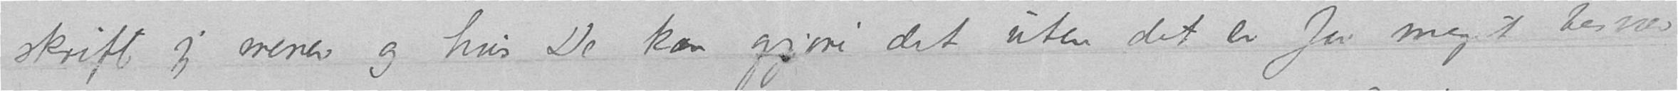skrift jeg mener og hvis De kan gjøre det uten det er for meget besvær
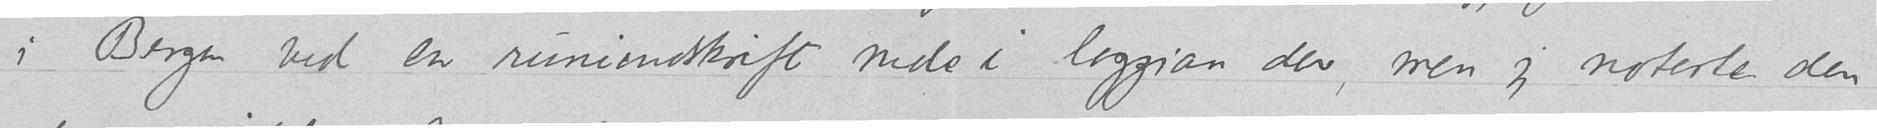i Bergen ved en runeindskrift nede i loggian der, men jeg noterte den
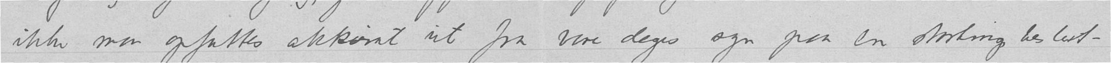ikke maa opfattes akkurat ut fra vore dages syn paa en stortingbeslut-
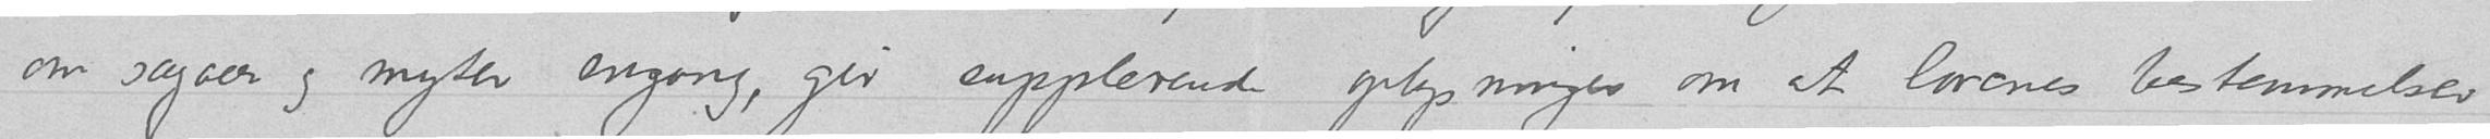om sagaer og myter engang, gir supplerende oplysninger om at lovenes bestemmelser
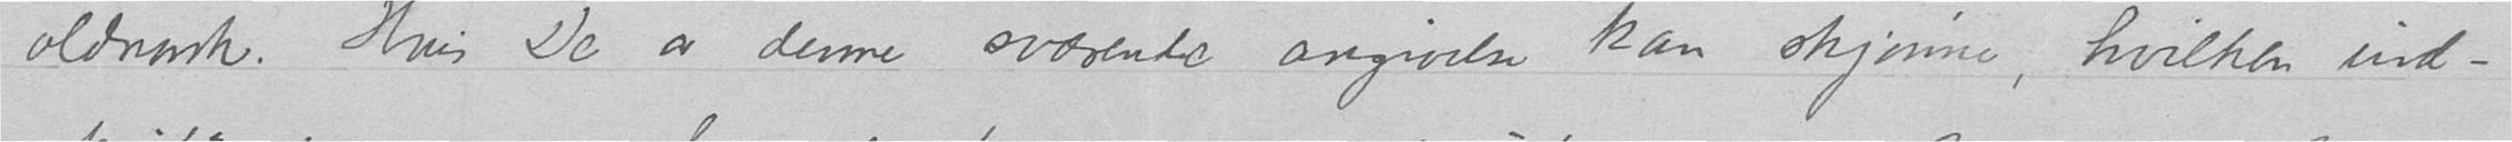oldnorsk. Hvis De av denne svævende angivelse kan skjønne, hvilken ind-
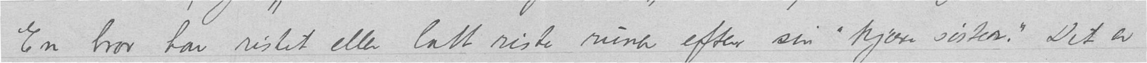En bror har ristet eller latt riste runer efter sin "kjære søster." Det er
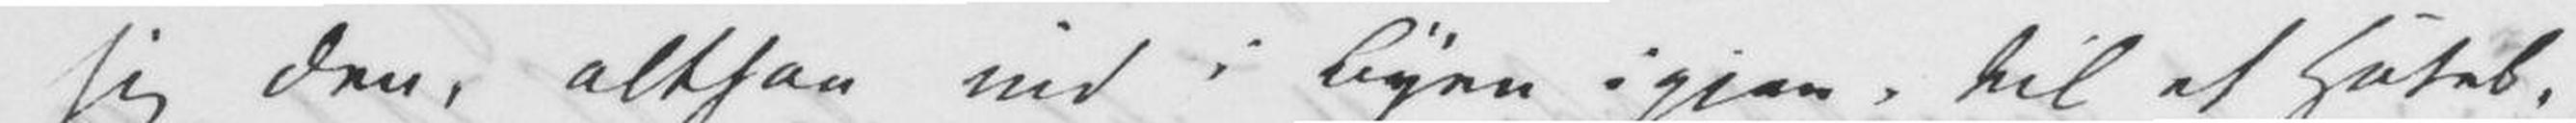sig den, altsaa ind i Byen igjen, til et Hotel,
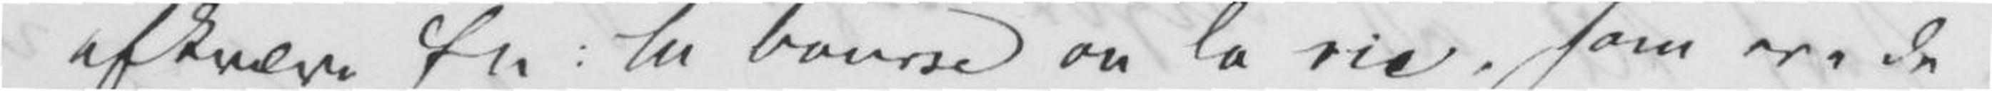afkræver En: tu bourse ou la vie, som ere de
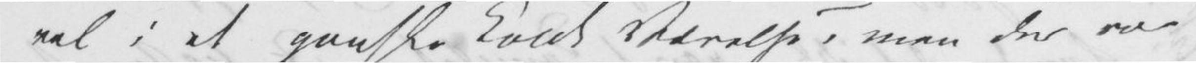vel i et ganske koldt Værelse, men der var
